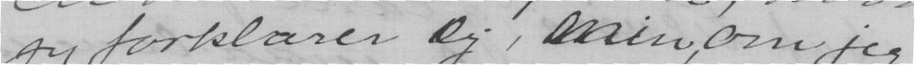jeg forklarer Dig, Min, om jag
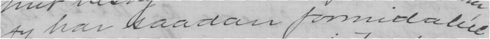jeg har saadan formidabel
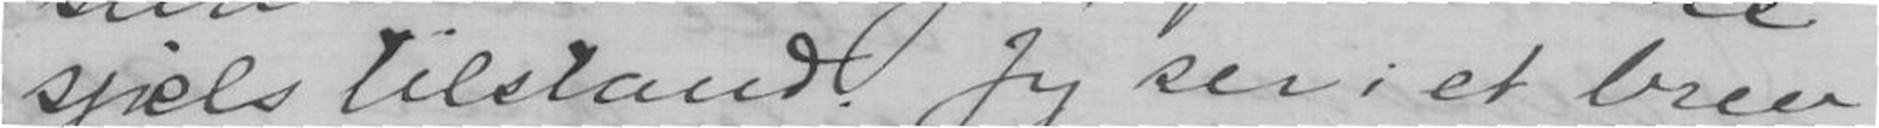sjiels tilstand. Jag ser i et brev
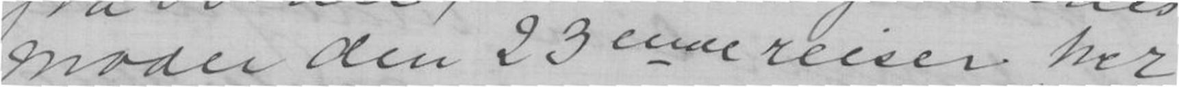moder den 23ende reiser her
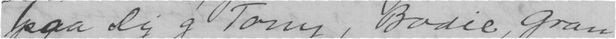paa Dig og Tony, Bodil, Gran
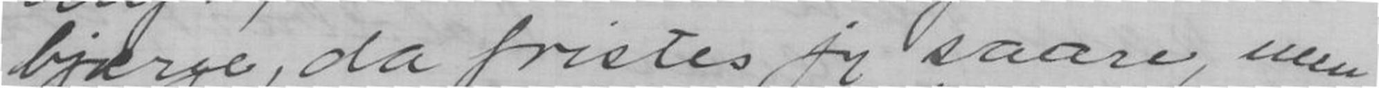bjærge, da fristes jeg saare, men
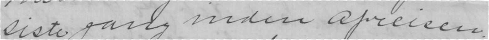siste gang inden Afreisen.
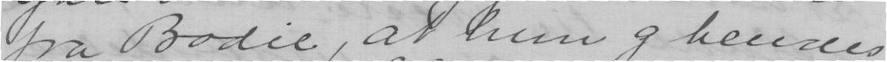fra Bodil, at hun og hendes
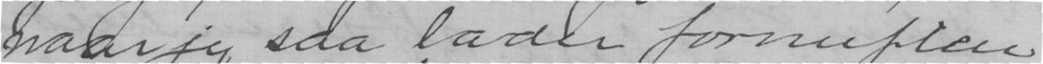naar jeg saa lader fornuften
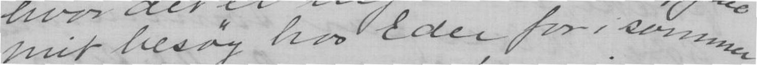mit besög hos Eder for i sommer
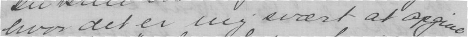hvor det er mig svært at opgive
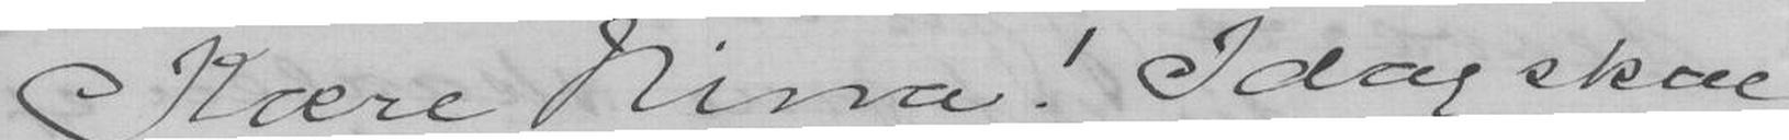Kære Nina! I dag skal
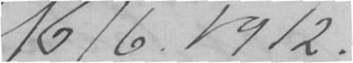16/6. 1912.
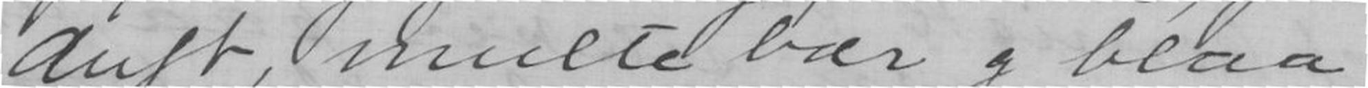duft, multebær og blaa
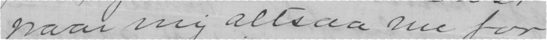naar mig altsaa nu for
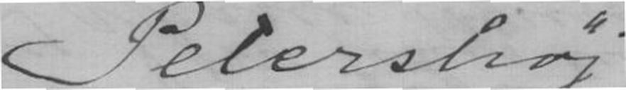Petershöj
